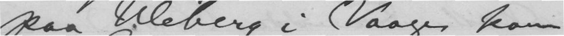paa Uleberg i Vaage kom
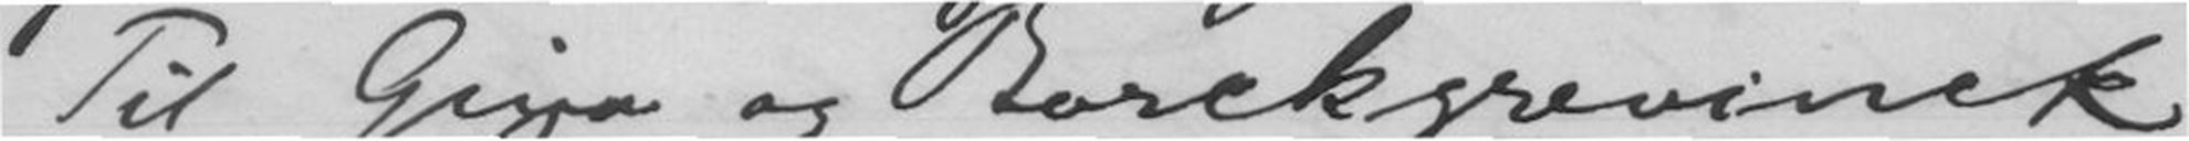Til Gina og Borckgrevinck
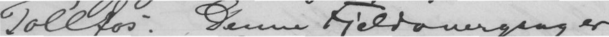til Pollfos. Denne Fjeldovergang er
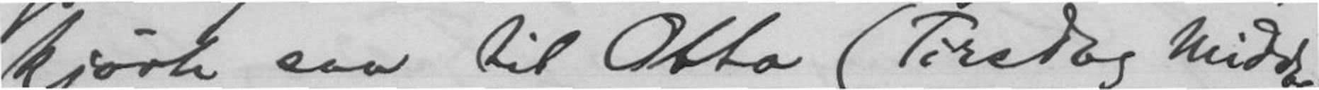kjørte saa til Otta (Tirsdag Middag)
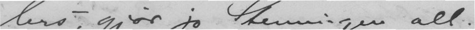lers, gjør jo Stemningen alt.
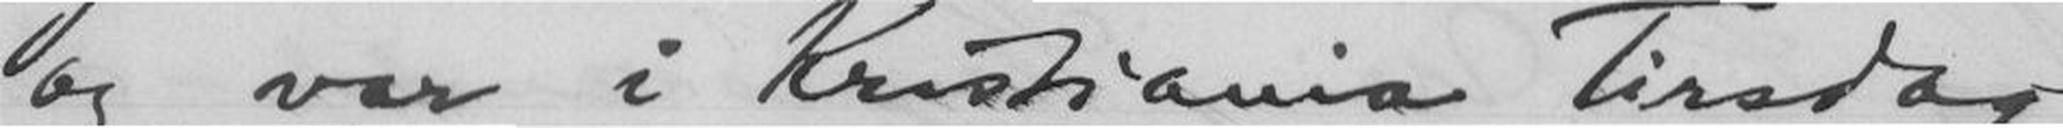og var i Kristiania Tirsdag
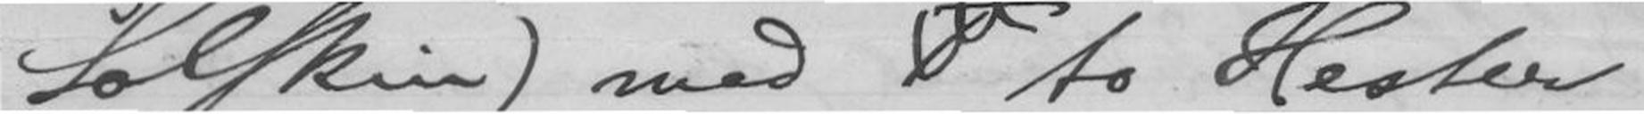Solskin) med T to Hester,
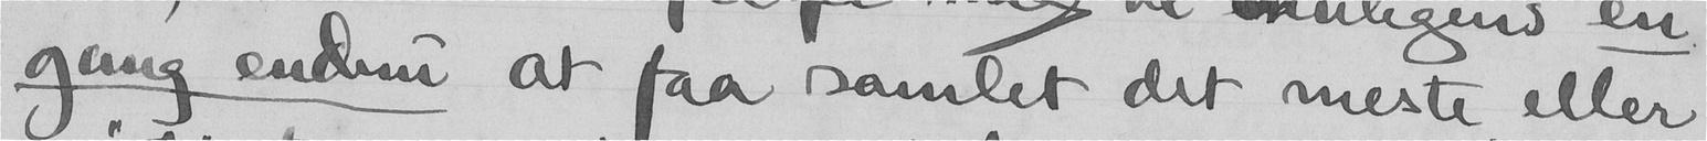gang endnu at faa samlet det meste eller
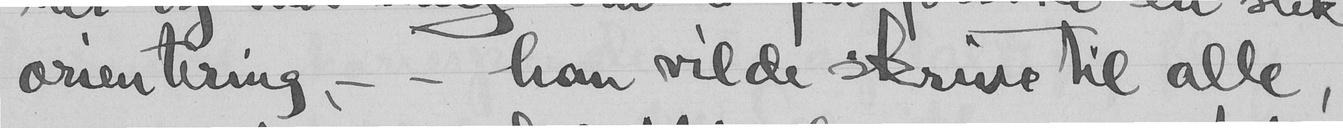orientering, - - han vilde skrives til alle,
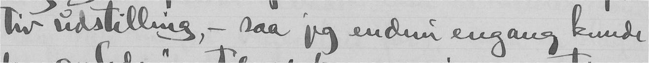tiv udstilling, - saa jeg endnu engang kunde
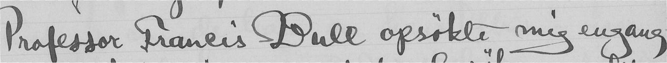Professor Francis Bull opsøkte mig engang
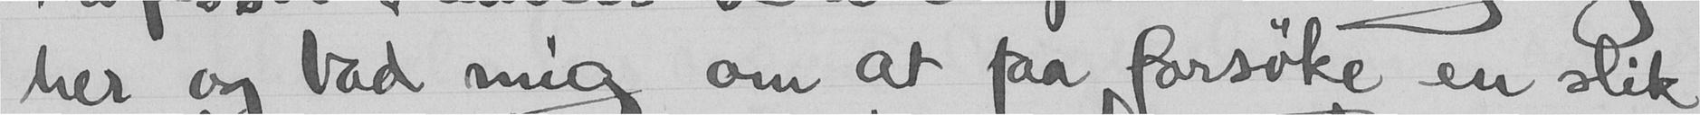her og bad mig om at faa forsøke en slik
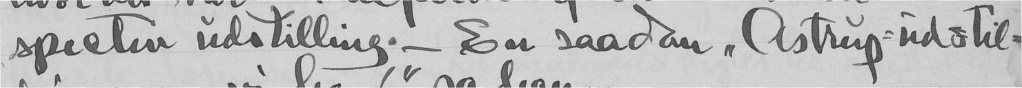spectiv udstilling. - En saadan "Astrup-udstil-
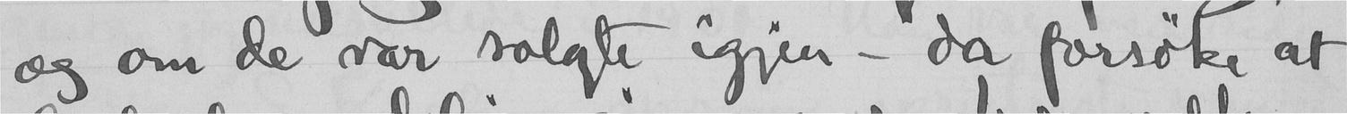og om de var solgte igjen - da forsøke at
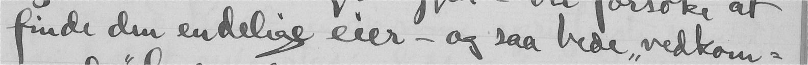finde den endelige eier - og saa bede "vedkom-
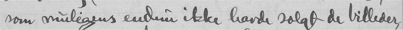som muligens endnu ikke havde solgt de billeder,
